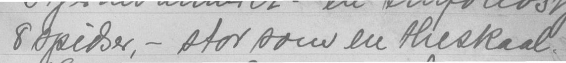8 spidser, - stor som en theskaal.
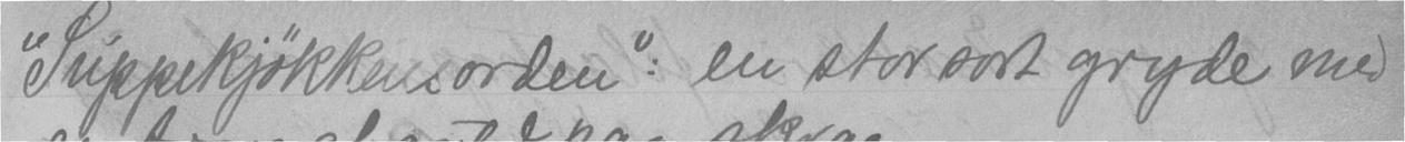"Suppekjøkkensorden": en stor sort gryde med
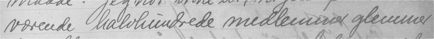værende halvhundrede medlemmer glemmer
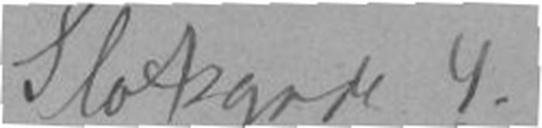Slotsgade 4.
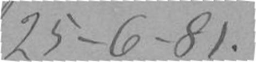25-6-81.
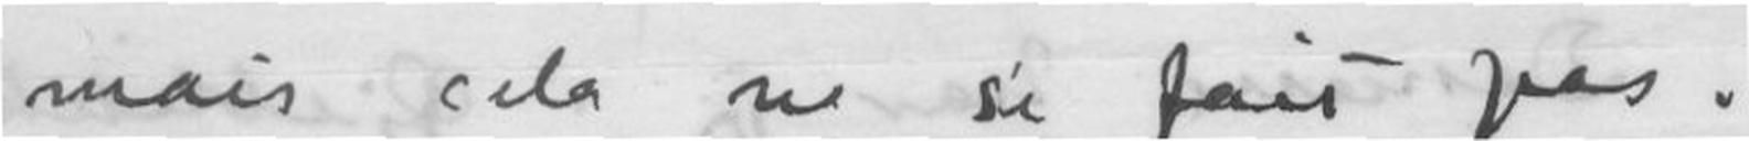mais cela ne si fait pas.
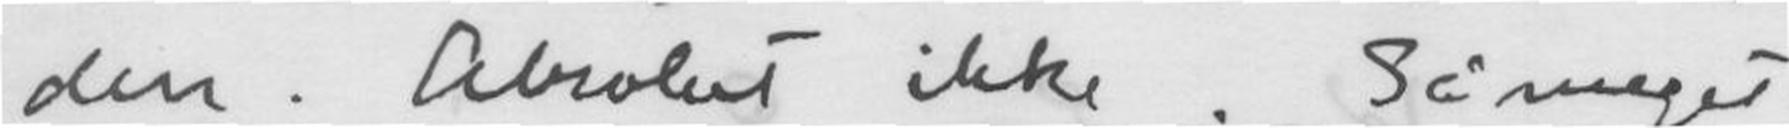den. Absolut ikke. Så meget
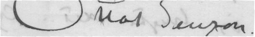Otto Benzon
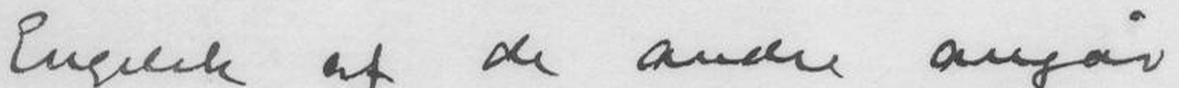Engelsk af de andre angår
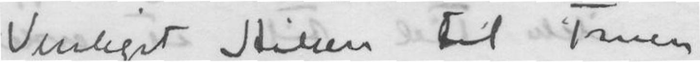Venligst Hilsen til Fruen
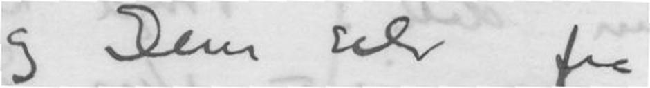og Dem selv fra
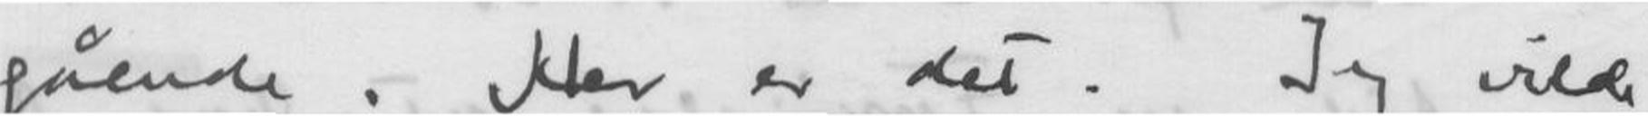gående. Her er det. Jeg vilde
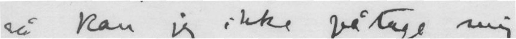så kan jeg ikke påtage mig
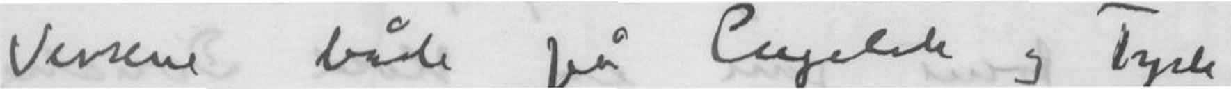Versene både på Engelsk og Tysk
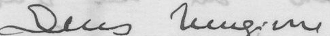Deres hengivne
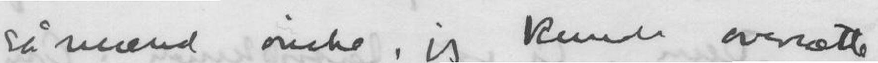så mænd ønske, jeg kunde oversætte
